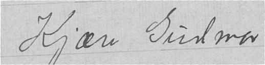Kjære Gudmar,
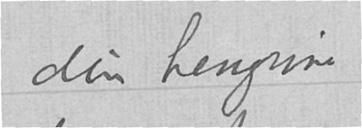din hengivne
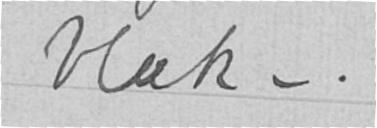blæk –.
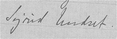Sigrid Undset.
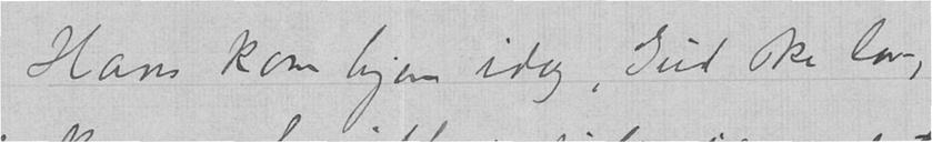Hans kom hjem idag, Gud ske lov,
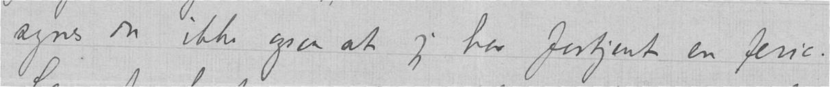syns du ikke ogsaa at jeg har fortjent en ferie.
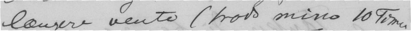længre vente (trods mins 10 Timer
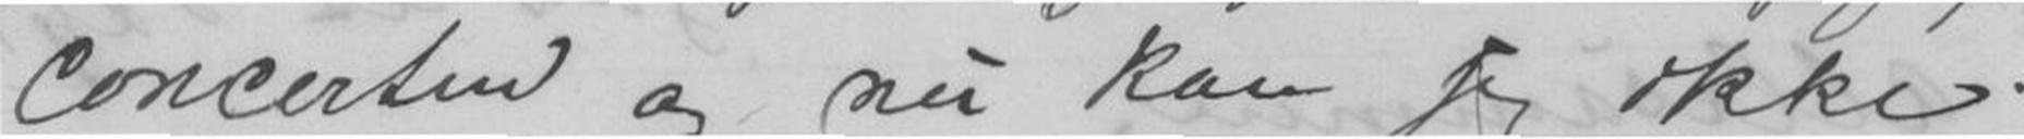concerten og nu kan jeg ikke
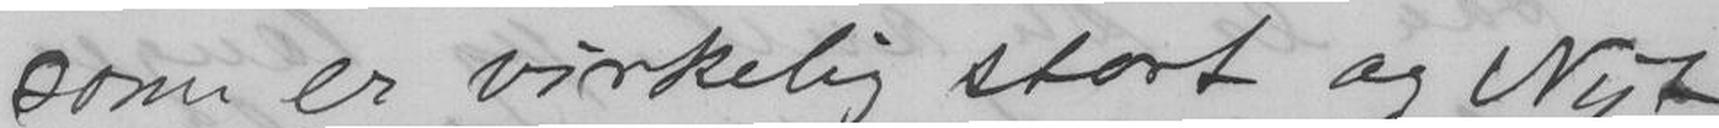som er virkelig stort og Nyt
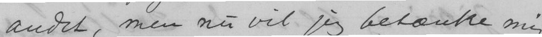andet, men nu vil jeg betænke
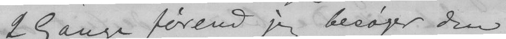mig 2 Gange førend jeg besøger din
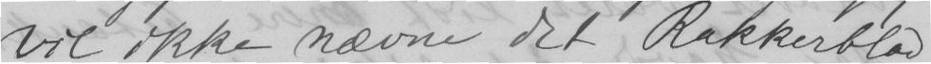vil ikke nænvne det Rakkerblad
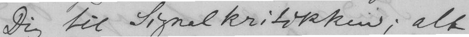Dig til Signalkritikken; alt
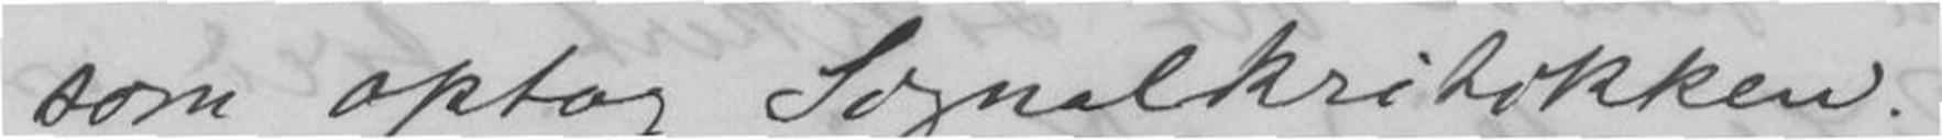som optog Signalkritikken.
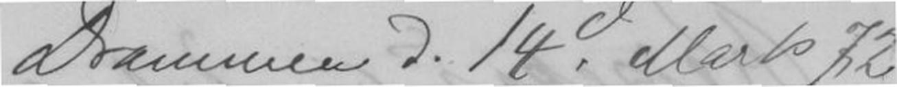Drammen d. 14d Marts 72
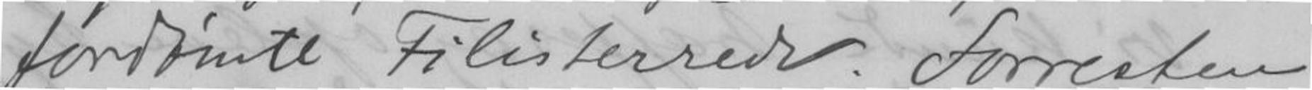fordømte Filisterred. Forresten
